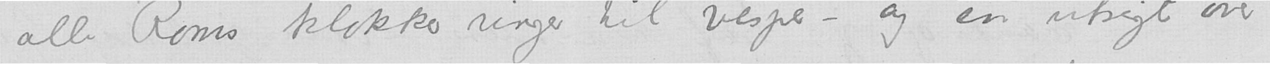alle Roms klokker ringer til vesper - og en utsigt over
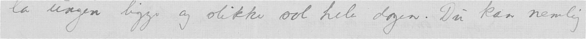la ungen ligge og slikke sol hele dagen. Du kan nemlig
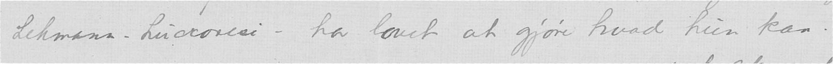Lehmann-Lucronesi - har lovet at gjøre hvad hun kan.
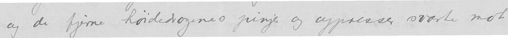og de fjerne høidedragenes pinjer og cypresser svarte mot
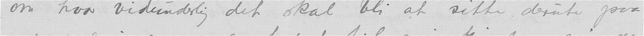om hvor vidunderlig det skal bli at sitte derute paa
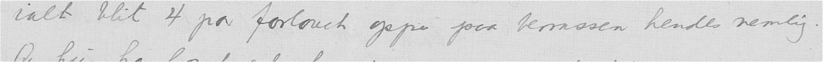ialt blit 4 par forlovet oppe paa terrassen hendes nemlig.
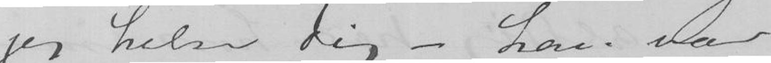jeg hilse Dig - han var
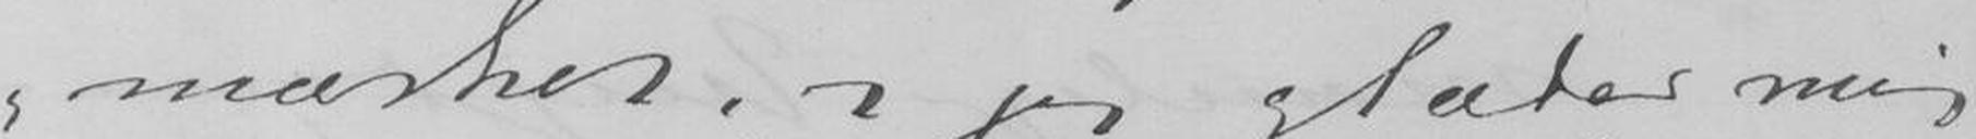mærket, og jeg glæder mig
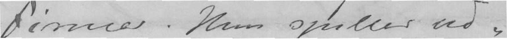Firma. Hun spiller ud-
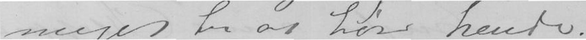meget til at høre hende.
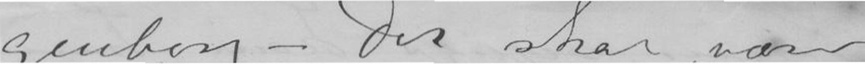genberg - Det skal være
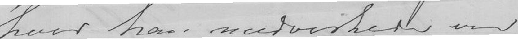hvor han medvirkede ved
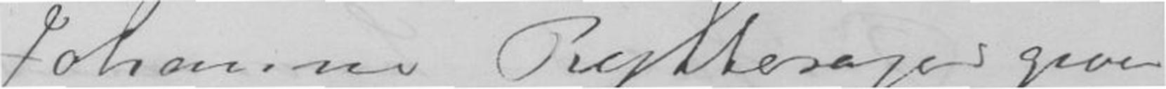Johanne Ryttersager giver
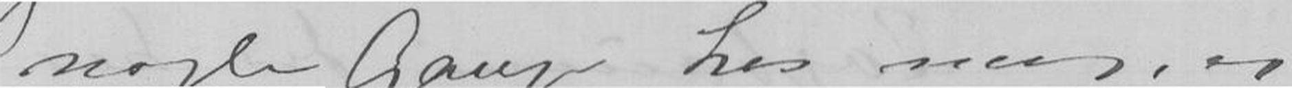nogle Gange hos mig, og
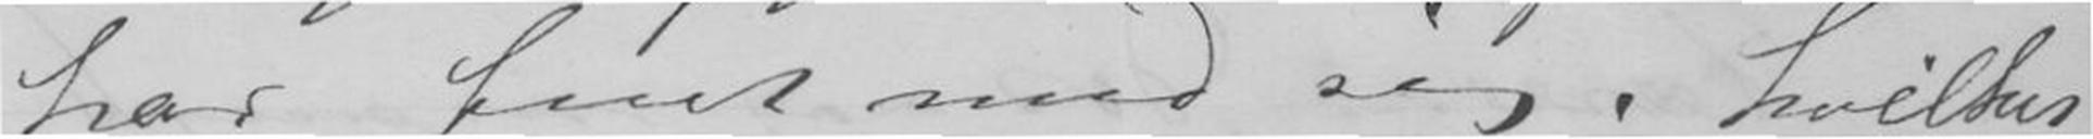har faaet med sig, hvilket
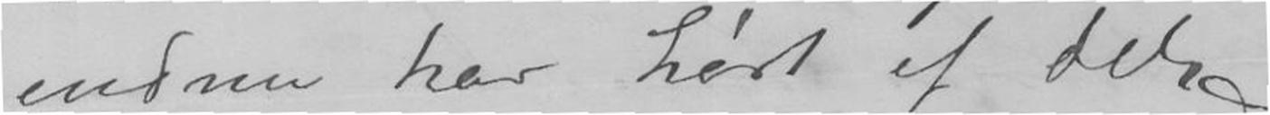endnu har hørt af det
